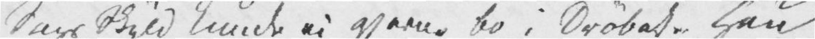Sags Skyld kunde vi gjerne bo i Drøbak. Han
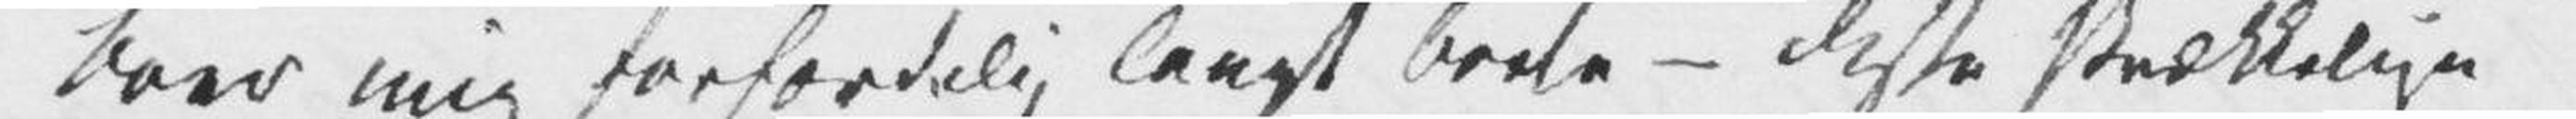boer mig forfærdelig langt borte – disse skrækkelige
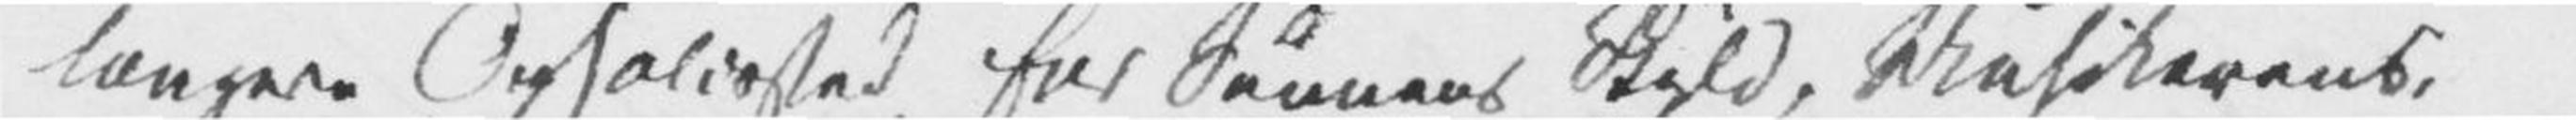længere Opholdssted for Sønnens Skyld, Musikerens,
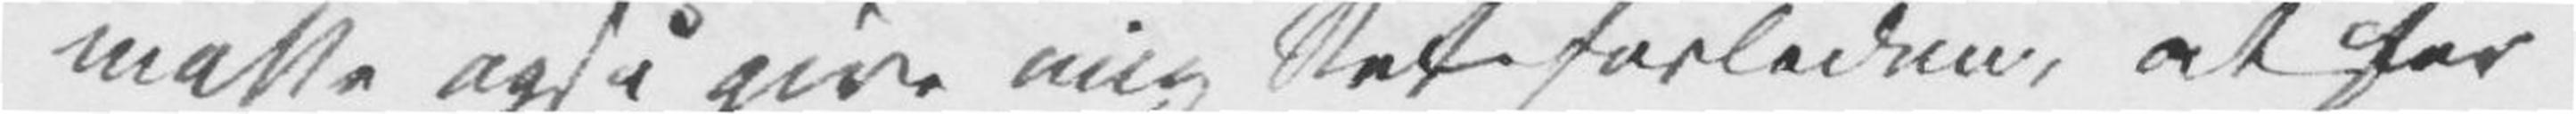måtte også give mig Ret forleden, at for
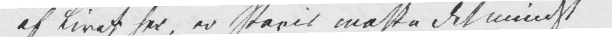af Livet her, er Paris måske det mindst
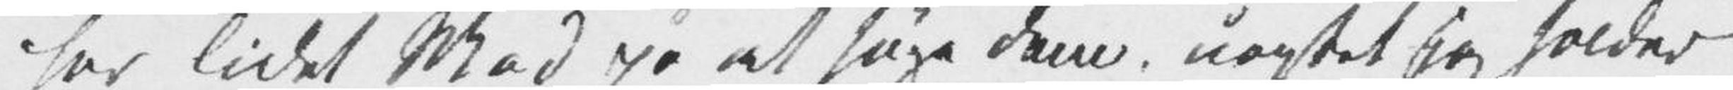har lidet Mod på at søge dem, uagtet jeg holder
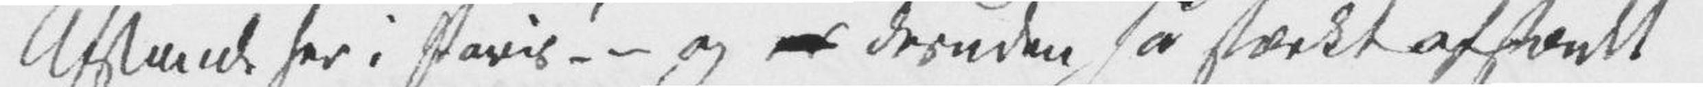Afstande her i Paris! – og er desuden så stærkt afstænkt
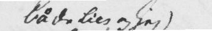både Lies og jeg
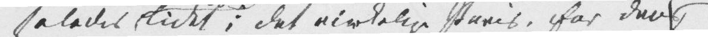således lidet i det virkelige Paris, for den
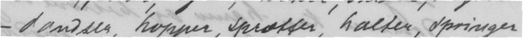- dandser, hopper, sprætter, halter, springer
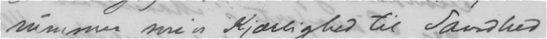rummer min Kjærlighed til Sandhed
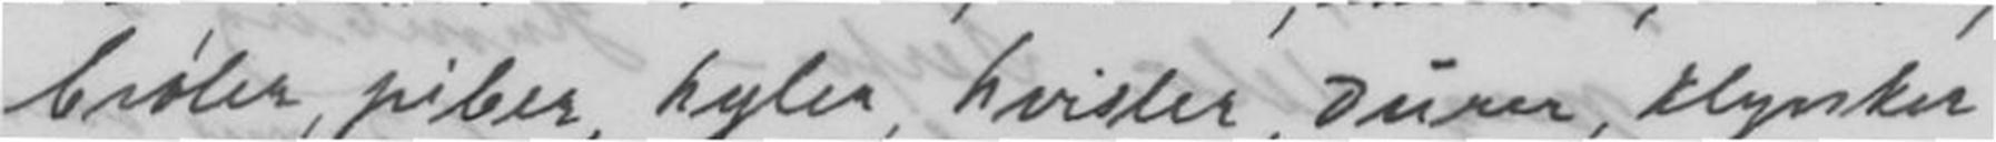brøler, piber, hyler, hvisler, durer, klynker
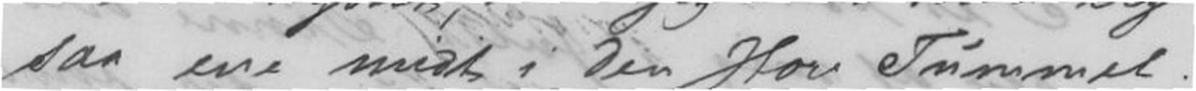saa ene midt i den store Tummel.
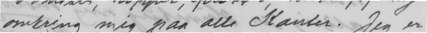omkring mig paa alle Kanter. Jeg er
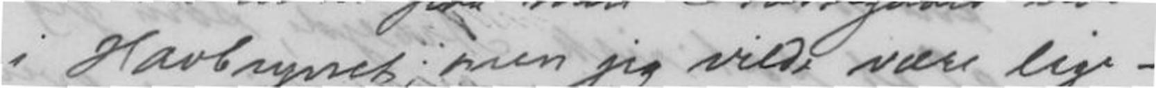i Havbrynet, men jeg vilde være lige-
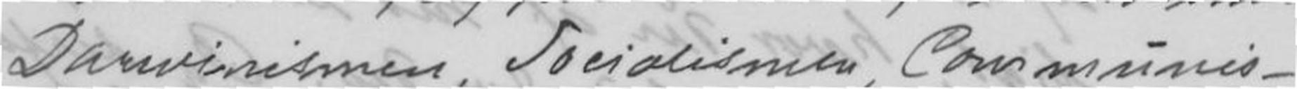Darwinismen, Socialismen, Communis-
